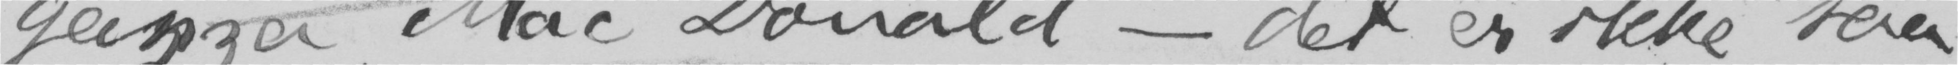genza, Mac Donald – det er ikke saa
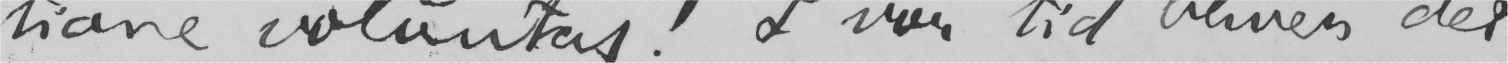tione voluntas! I vor tid bliver det
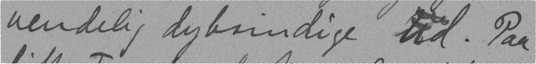uendelig dybsindige ud. Paa
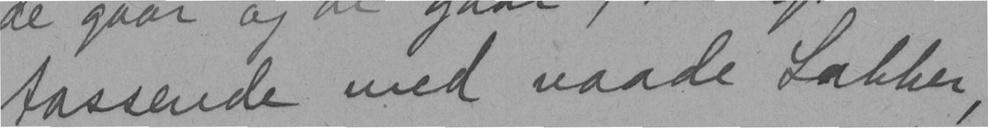tassende med vaade Labber,
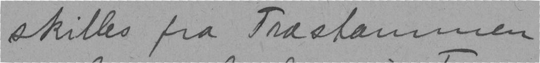skilles fra Træstammen
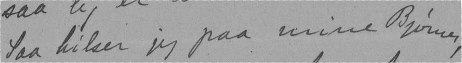Saa hilser jeg paa mine Bjørner,
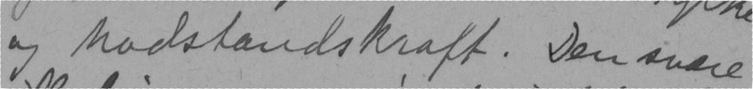og Modstandskraft. Den svære
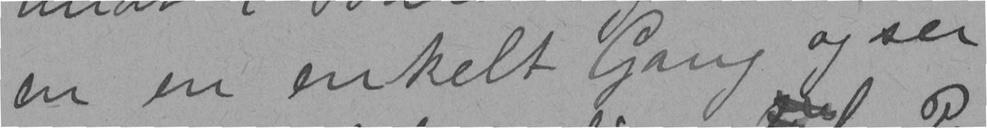en en enkelt Gang og ser
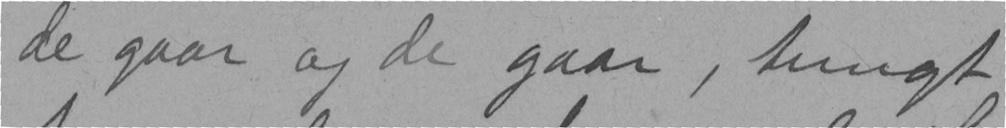de gaar og de gaar, tungt
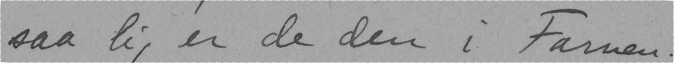saa lig er de den i Farven.
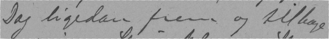Dag ligedan frem og tilbage
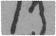13
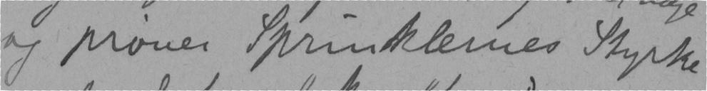og prøver sprinklernes Styrke
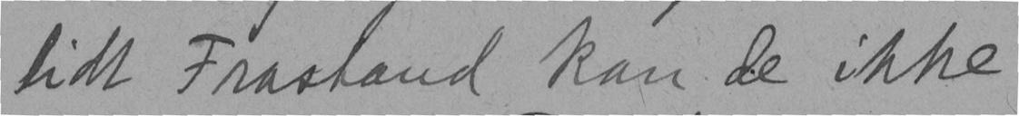lidt Frastand kan de ikke
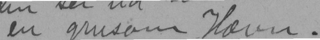en grusom Hævn.
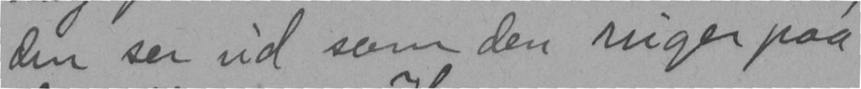den ser ud som den ruger paa
